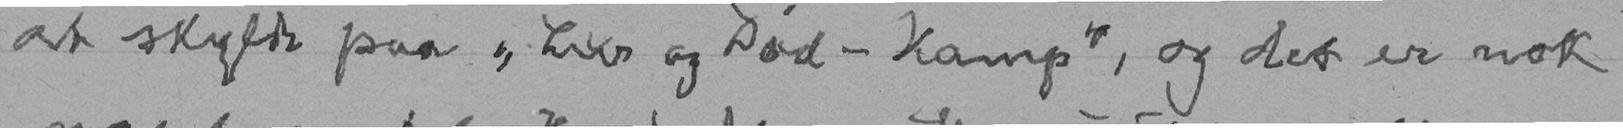at skylde paa "Liv og Død - Kamp", og det er nok
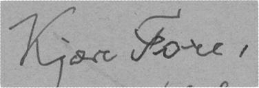Kjære Tore,
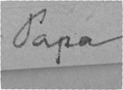Papa
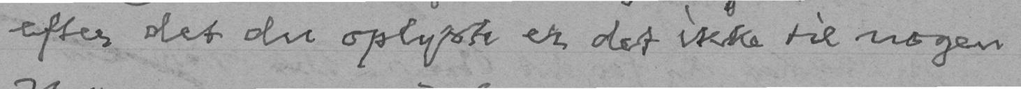efter det du oplyste er det ikke til nogen
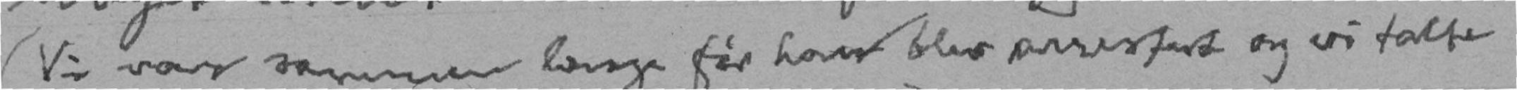(Vi var sammen længe før han blev arrestert og vi talte
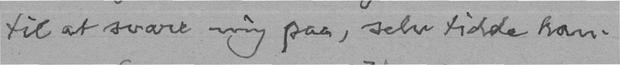til at svare mig paa, selve tidde han.
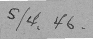5/4. 46.
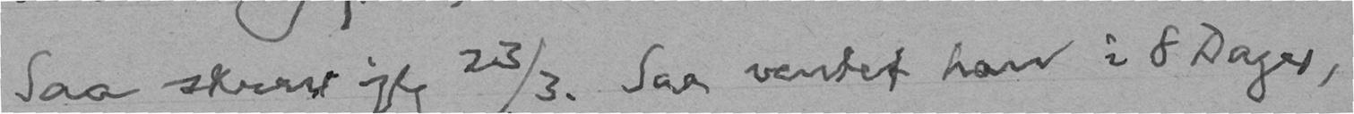Saa skrev jeg 23/3. Saa ventet han i 8 Dager,
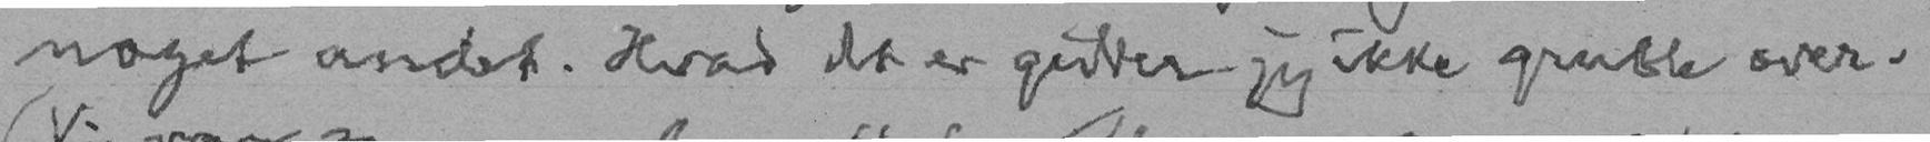noget andet. Hvad det er gidder jeg ikke gruble over.
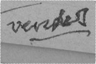vendes
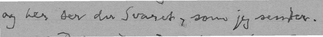og her ser du Svaret, som jeg sender.
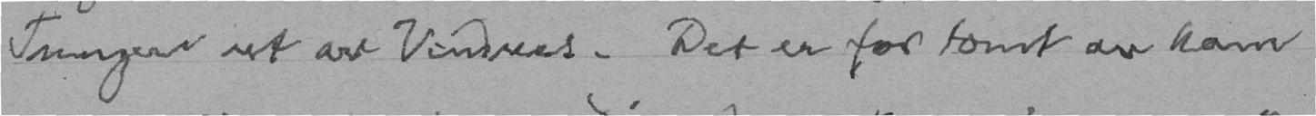Tungen ut av Vinduet. Det er for tomt av ham
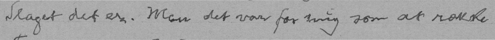Slaget det er. Men det var for mig som at række
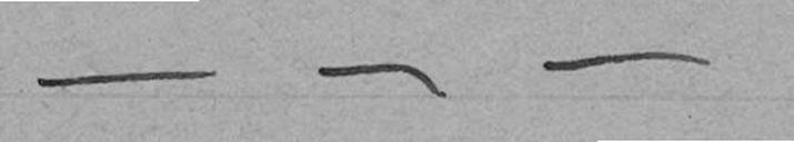- - -
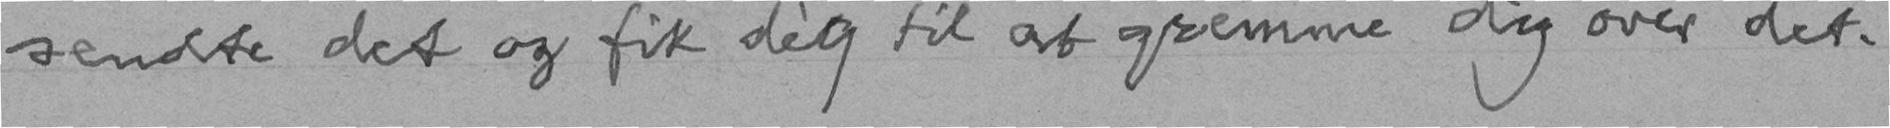sendte det og fik dig til at gremme dig over det.
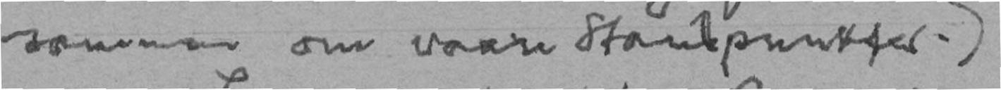sammen om vaare Standpunkter.)
